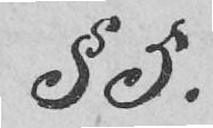§ 5.
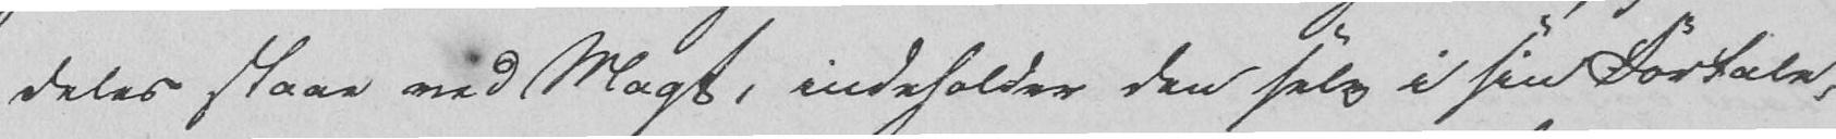deles staar ved Magt, indeholder den selv i sin Fortale,
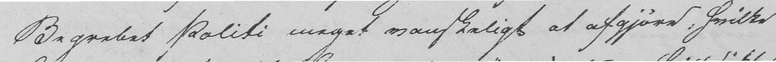Begrebet Politi meget vanskeligt at afgjøre, hvilke
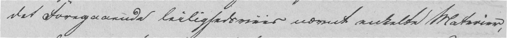det Foregaaende leilighedsviis nævnt enkelte Materier,
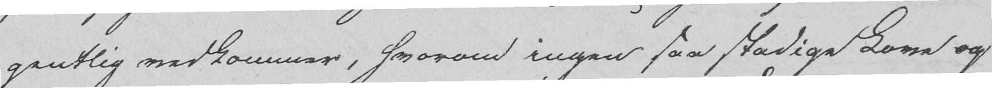gentlig vedkommer, hvorom ingen saa stadige Love og
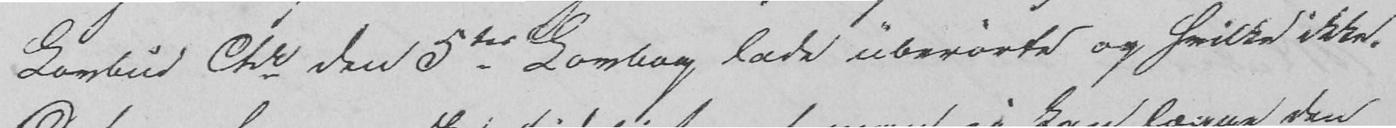Lovbud Chr den 5tes Lovbog lade uberørte og hvilke ikke.
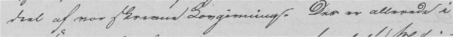deel af vor skrevne Lovgivning. Der er allerede i
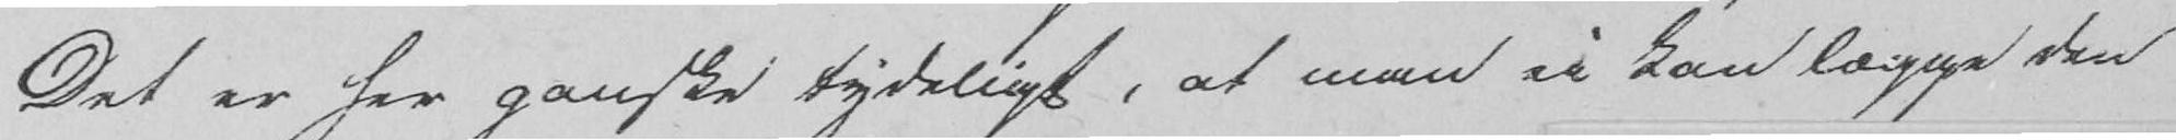Det er her ganske tydeligt, at man ei kan lægge den
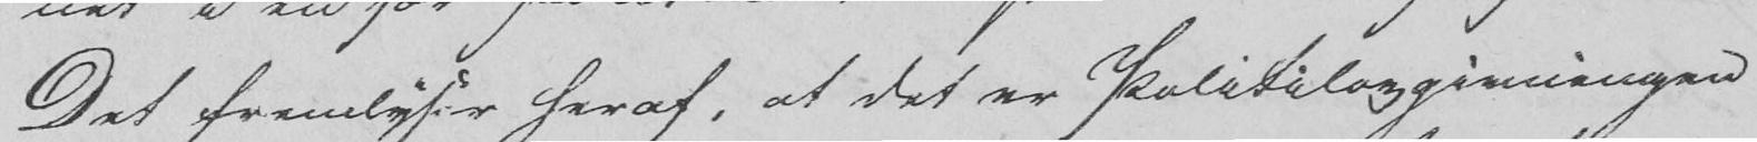Det fremlyser heraf, at det er Politilovgivningen
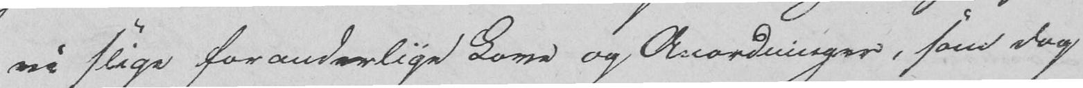vi slige foranderlige Love og Anordninger, som dog
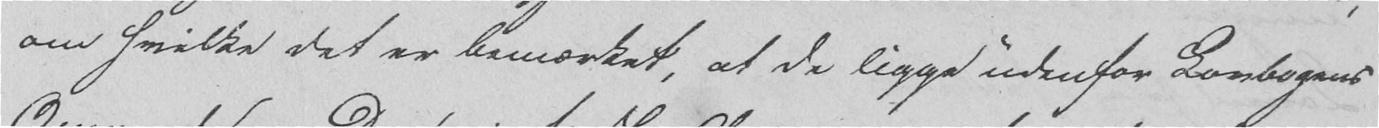om hvilke det er bemærket at de ligge udenfor Lovbogens
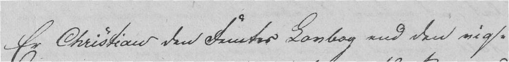Er Christian den Femtes Lovbog end den vig-
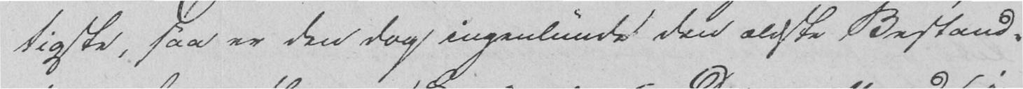tigste, saa er den dog ingenlunde den ældste Bestand-
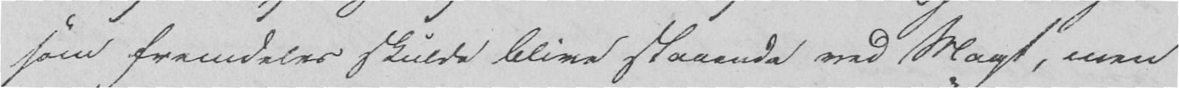som fremdeles skulde blive staaende ved Magt, men
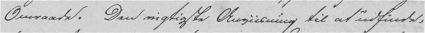Omraade. Den vigtigste Anviisning til at udfinde,
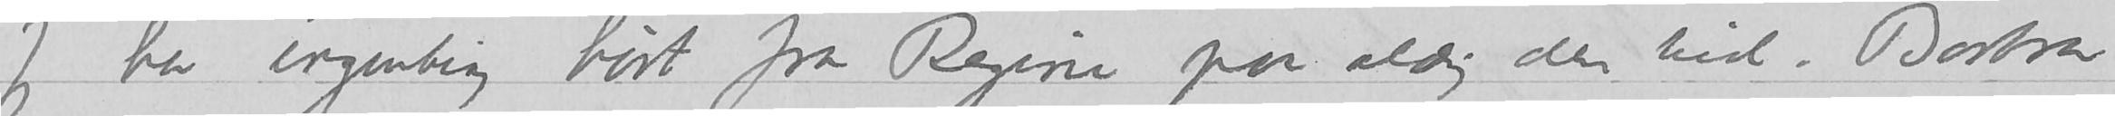Jeg har ingenting hørt fra Regine paa aldrig den tid.  Barbra
